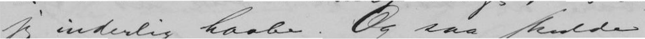jeg inderlig haabe. Og saa skulde
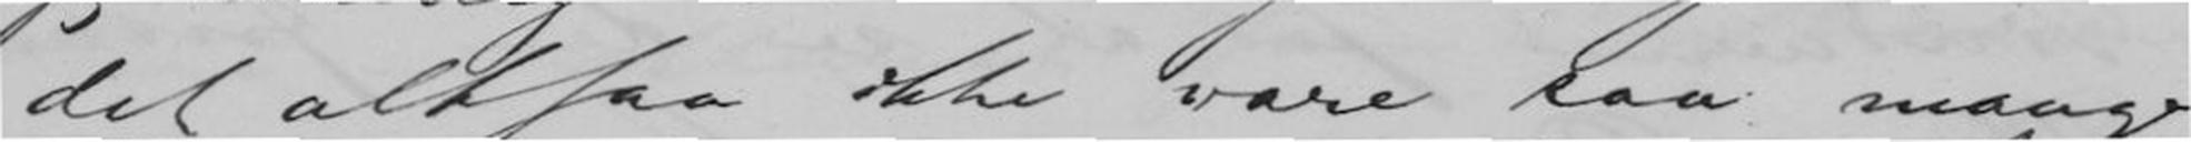det altsaa ikke være saa mange
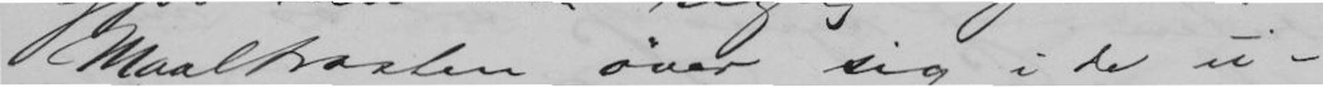Maaltrosten øver sig i de u-
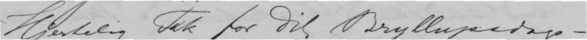Hjertelig Tak for Dit Bryllupsdags-
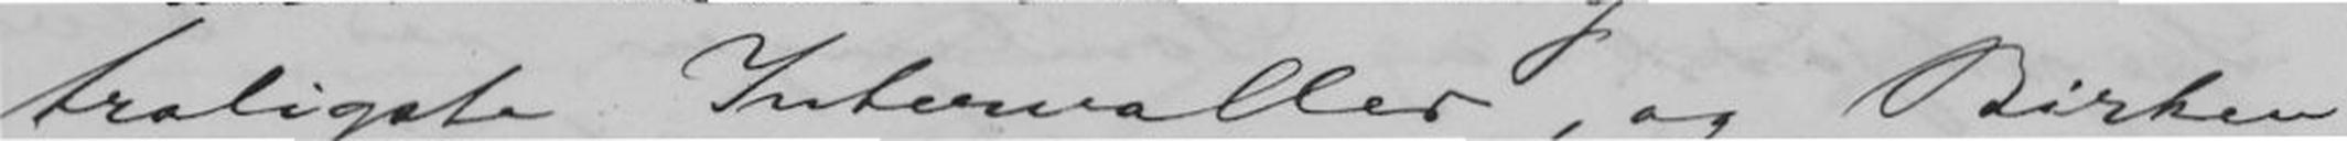troligste Intervaller, og Birken
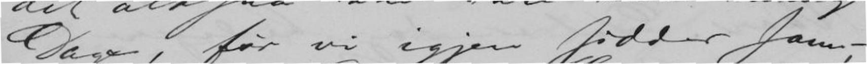Dage, før vi igjen sidder sam-
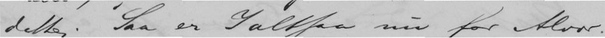dette. Saa er I altsaa nu for Alvor
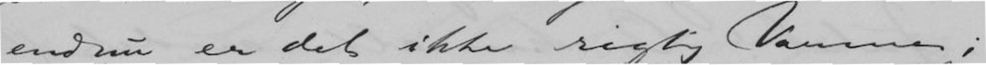endnu er det ikke rigtig Varme;
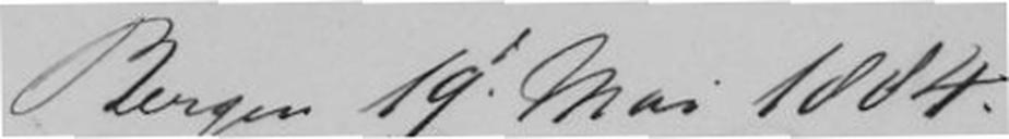Bergen 19de Mai 1884.
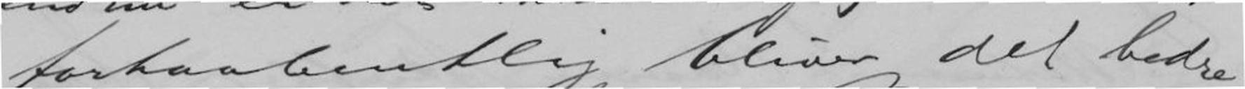forhaabentlig bliver det bedre
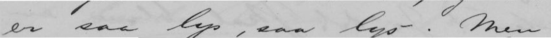er saa lys, saa Lys. Men -
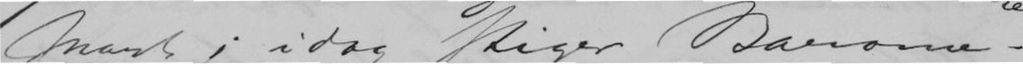snart; idag stiger Barome-
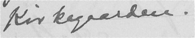Kirkegaarden.
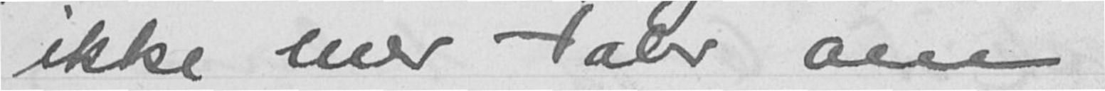ikke mer taler om
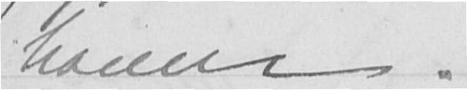haven. -
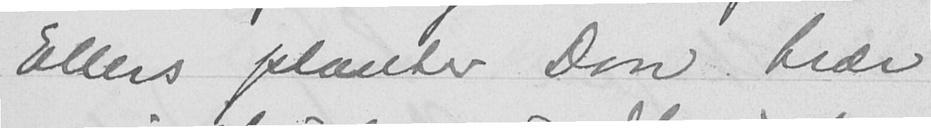Ellers planter Don trær
